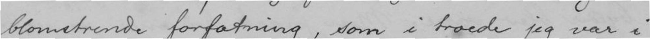blomstrende forfatning, som i troede jeg var i
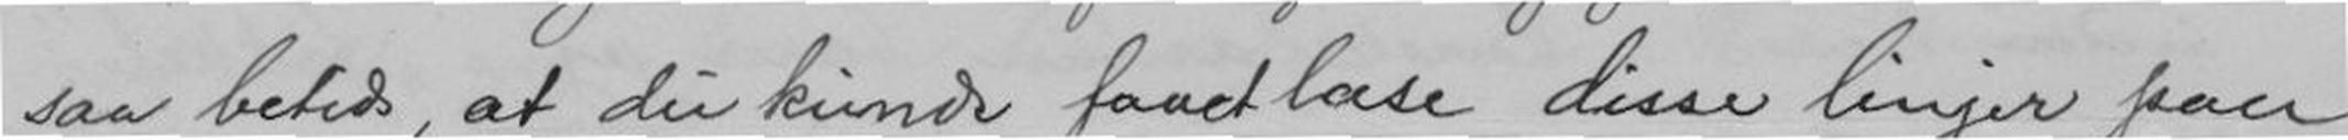saa betids, at du kunde faaetlæse disse linjer paa
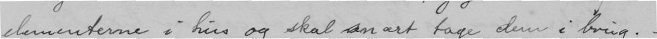elementerne i hus og skal snart tage dem i brug. -
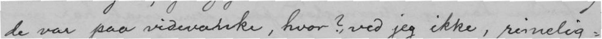de var paa videvanke, hvor?, ved jeg ikke, rimelig-
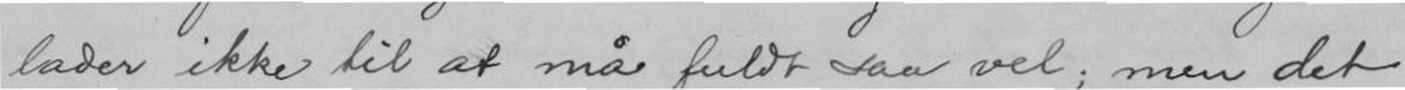lader ikke til at må fuldt saa vel; men det
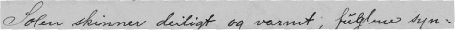Solen skinner deiligt og varmt, fuglene syn-
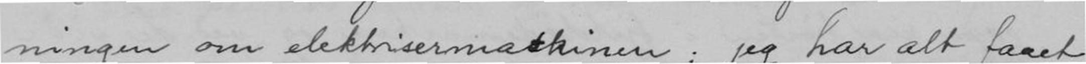ningen om elektrisermaskinen; jeg har alt faaet
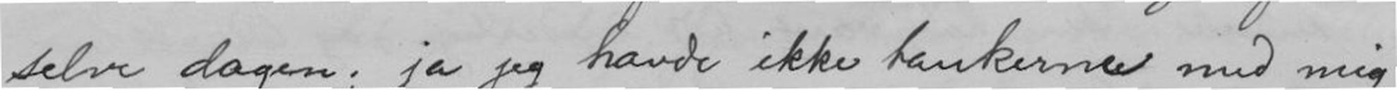selve dagen; ja jeg havde ikke tankerne med mig;
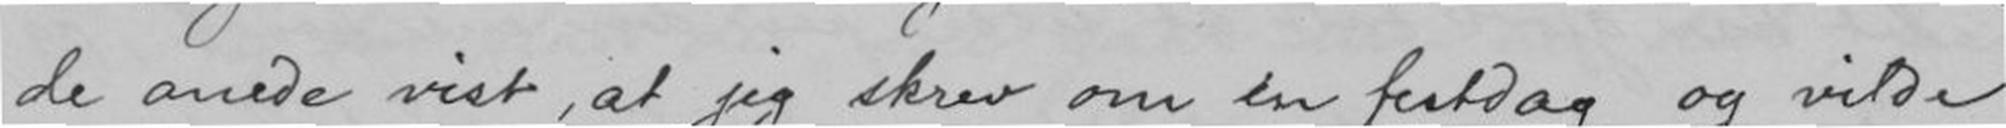de anede vist, at jeg skrev om en festdag og vilde
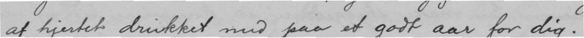af hjertet drukket med paa et godt aar for dig.
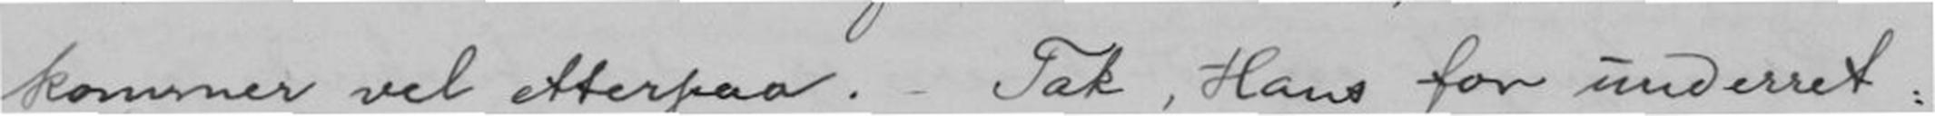kommer vel etterpaa. Ta, Hans for underret-
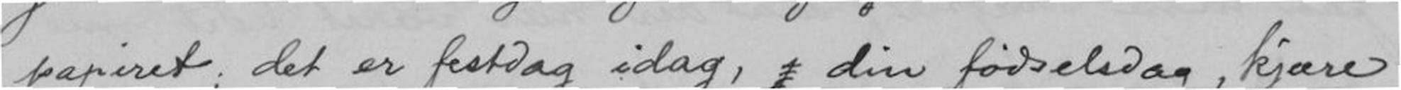papiret; det er festdag idag, din fødselsdag, kjære
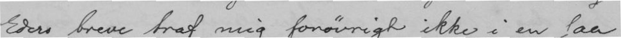Eders breve traf mig forøvrig ikke i en saa
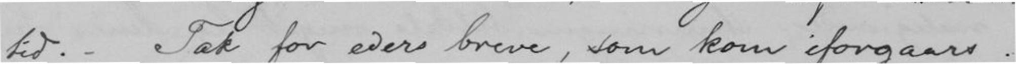tid. - Tak for eder breve, som kom iforgaars.
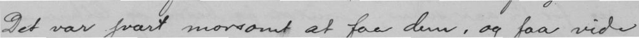Det var svært morsomt at faa den, og faa vide
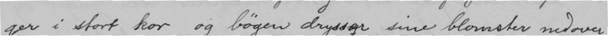ger i stort kor og bøgen drysser sine blomster nedover
